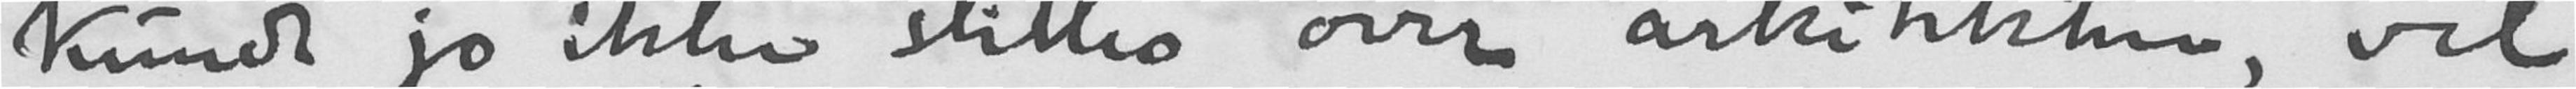kunde jo ikke stilles over arkitekten, vel
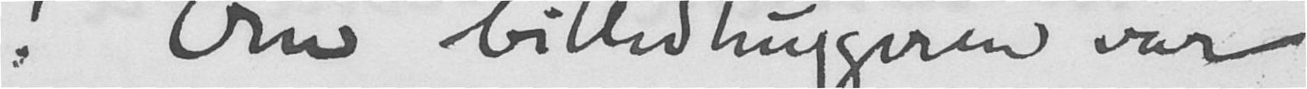! Om billedhuggeren var
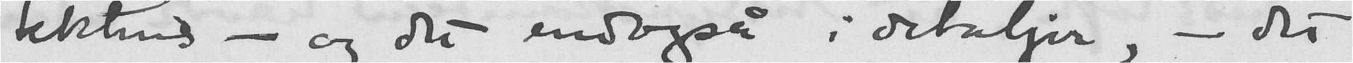tektens - og det endogså i detaljer, - det
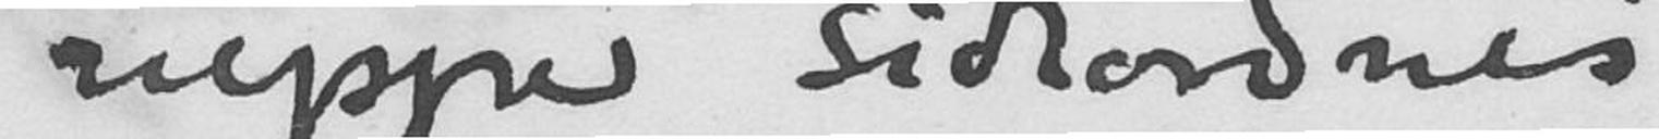neppe sideordnes
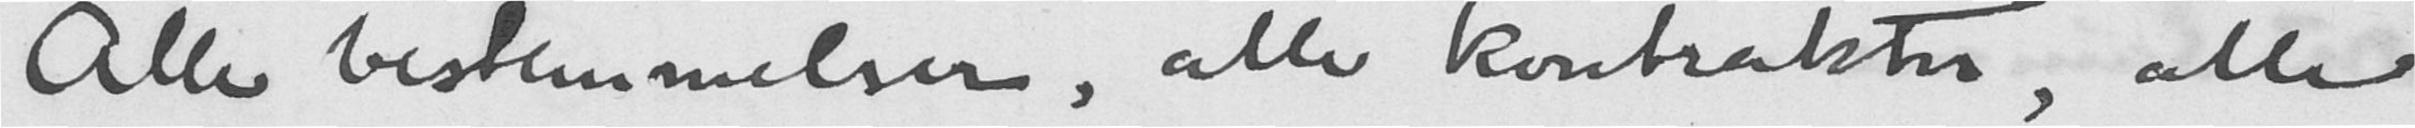Alle bestemmelser, alle kontrakter, alle
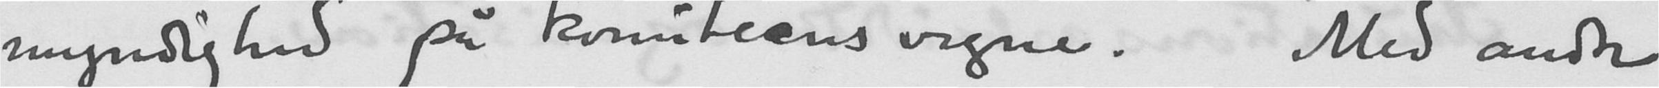myndighed på komiteens vegne. Med andre
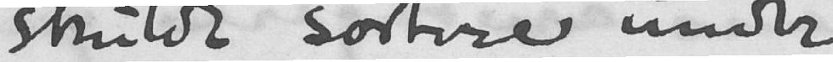skulde sortere under
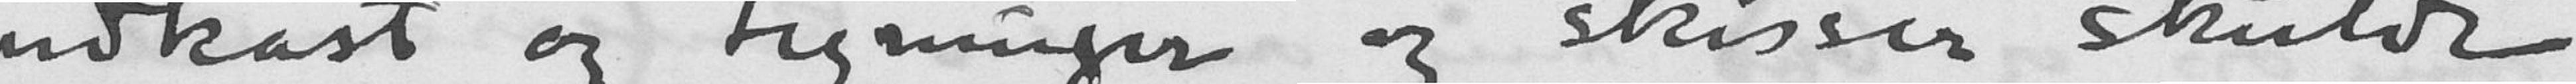udkast og tegninger og skisser skulde
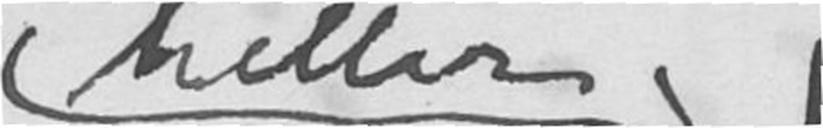heller
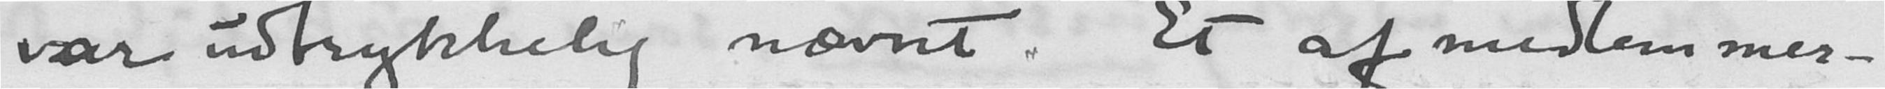var udtrykkelig nævnt. Et af medlemmer-
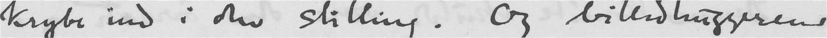krybe ind i den stilling. Og billedhuggeren
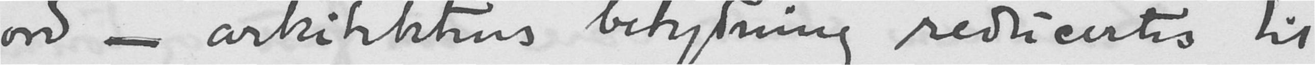ord - arkitektens betydning reducertes til
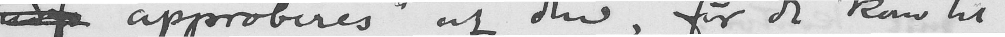udfra "approberes" af den, før de kom til
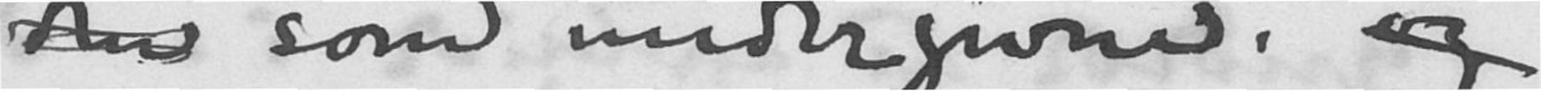den som undergivne. og
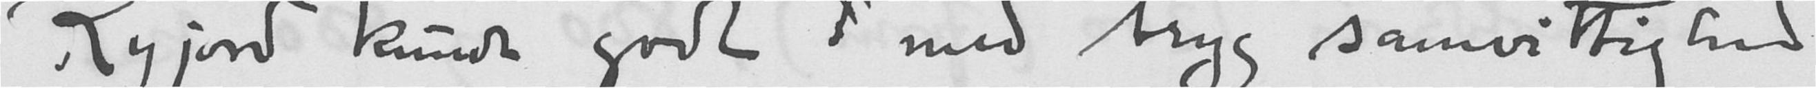Ryjord kunde godt med tryg samvittighed
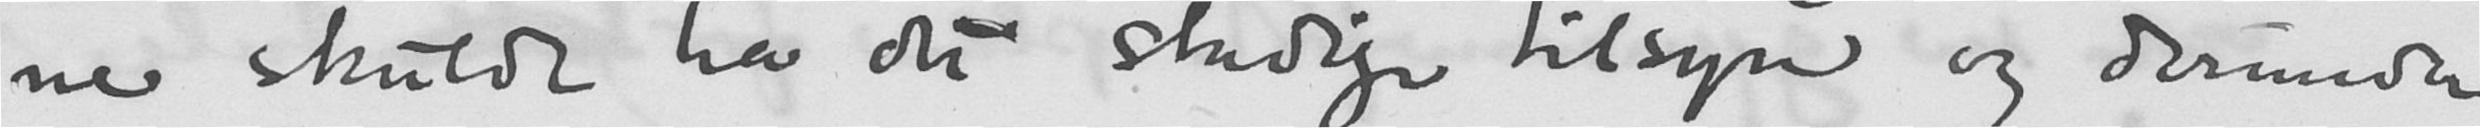ne skulde ha det stadig tilsyn og derunder
